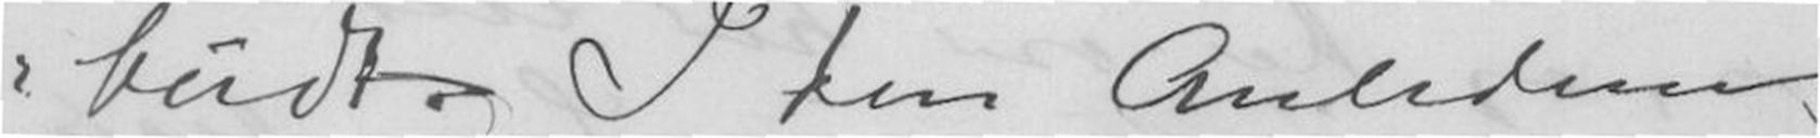budt. I den Anledning
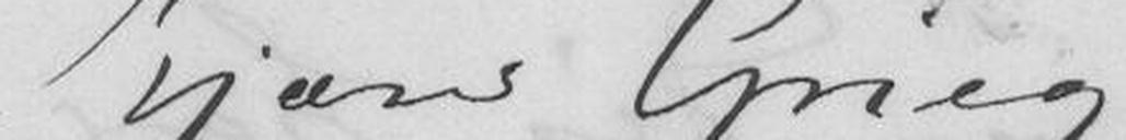Kjære Grieg
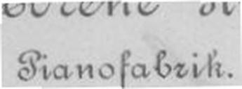Pianofabrik.
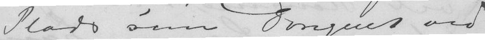Plads som Dirigent ved
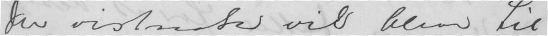Du vistnok vil blive til
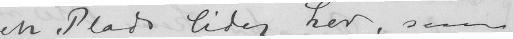en Plads ledig her, som
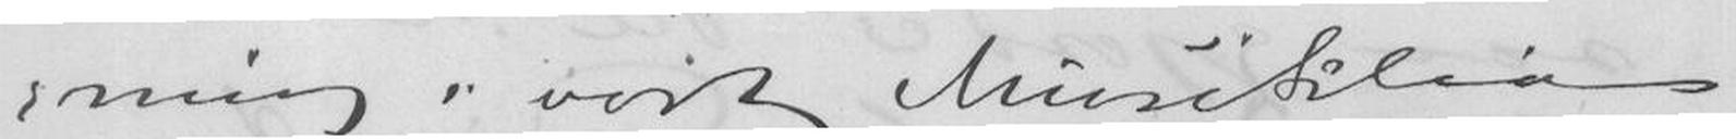ning i vort Musiklivs
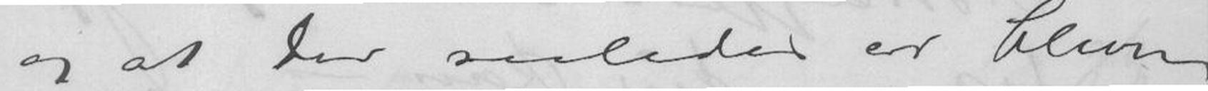og at der saaledes er bleven
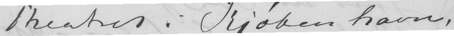Theatret i Kjøbenhavn,
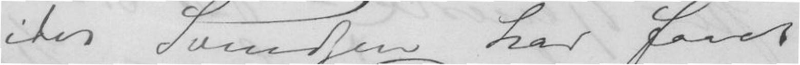idet Svendsen har faaet
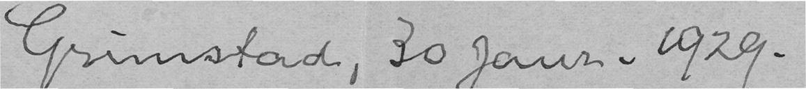Grimstad, 30 Janr. 1929.
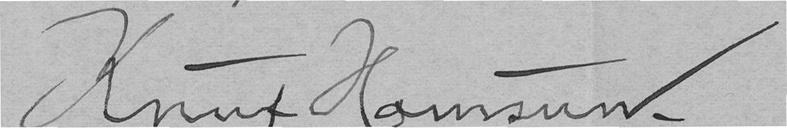Knut Hamsun.
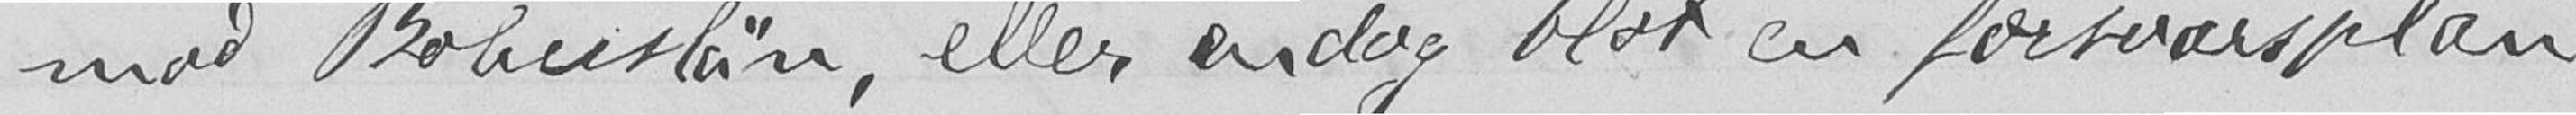mod Bohuslän, eller endog blot en forsvarsplan
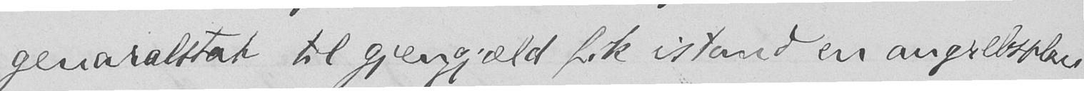genaralstab til gjengjæld fik istand en angrebsplan
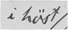i høst
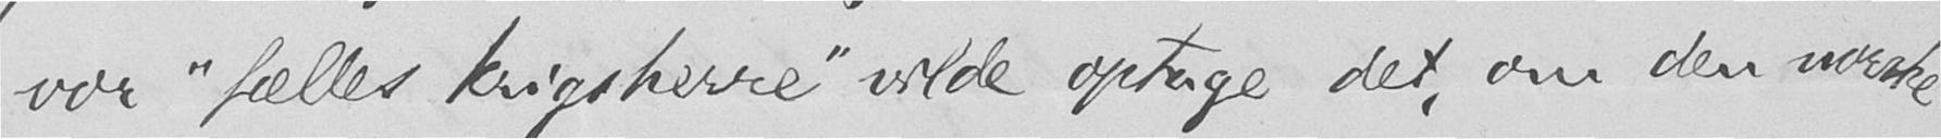vor "fælles krigsherre" vilde optage det, om den norske
